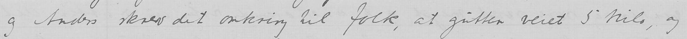og Anders skrev det omkring til folk, at gutten veiet 5 kilo, og
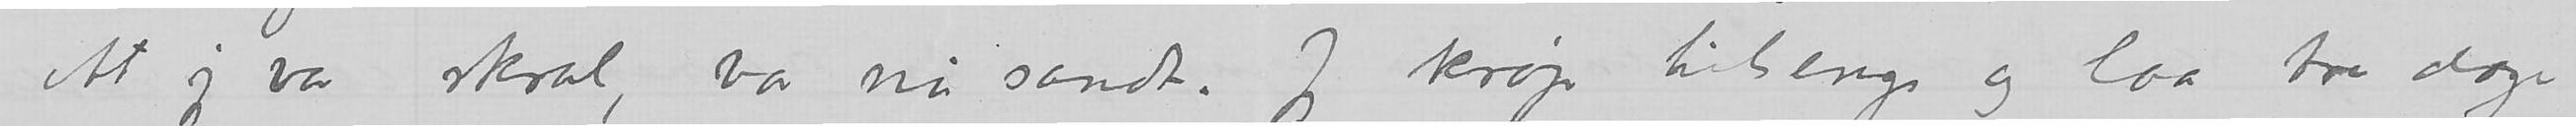At jeg var skral, var nu sandt. Jeg krøp til sengs og laa tre dager
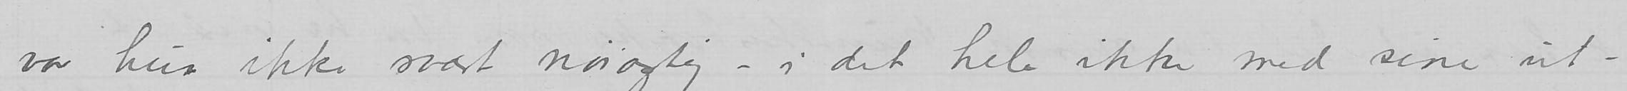var hun ikke svært nøiagtig – i det hele ikke med sine ut-
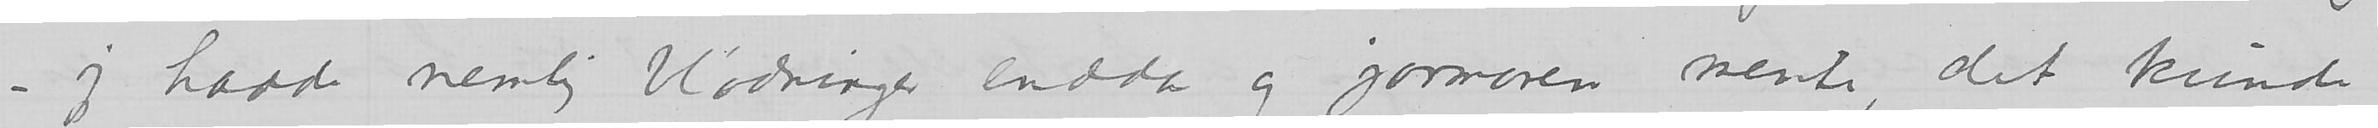– jeg hadde nemlig blødninger endda og jormoren mente, det kunde
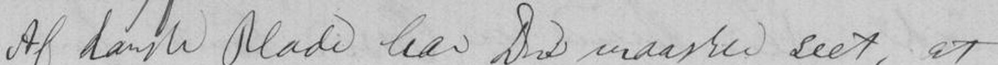Af dansk Blade har Du maaske seet, at
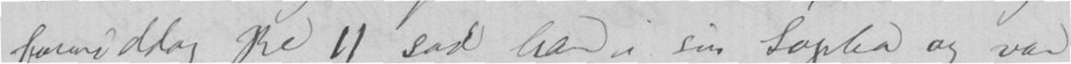formiddag Kl 11 sad han i sin Sopha og var
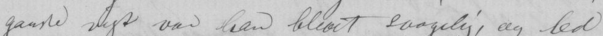ganske vist var han vlevet svagelig, og led
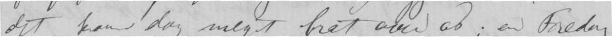det kom dog meget brat over os; en Fredag
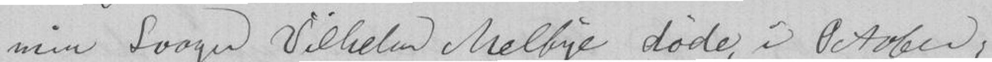min Svoger Vilhelm Melbye døde, i October,
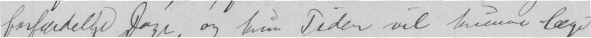forfærdelige Dage, og kun Tiden vil kunne læge
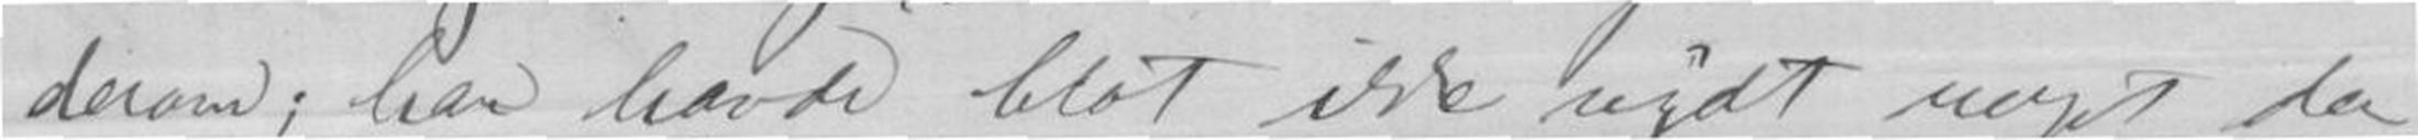derom; han havde blot ikke nydt meget den
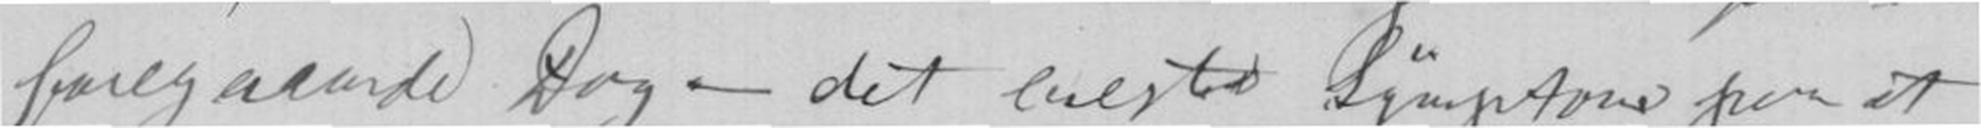foregaaende Dag - det lureste Symptom paa at
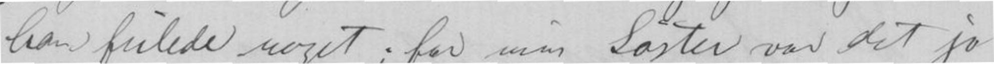han feilede noget; for min Søster var det jo
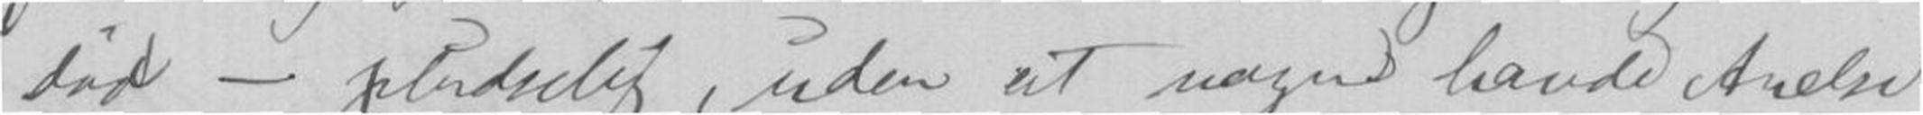død - pludselig, uden at nogen havde Anelse
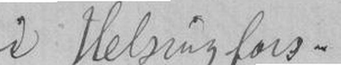i Helsingfors.
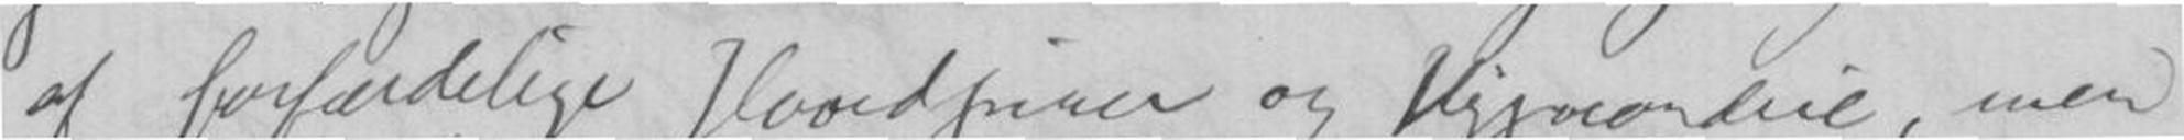af forfærdelige Hovedpiner og Hypocondrie, men
